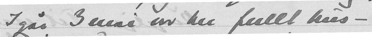Igår 3 mai var her fullt hus -
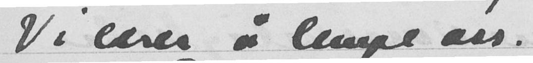Vi lærer å lempe oss.
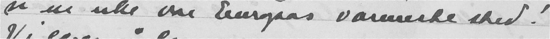vi en uke var Europas varmeste sted!
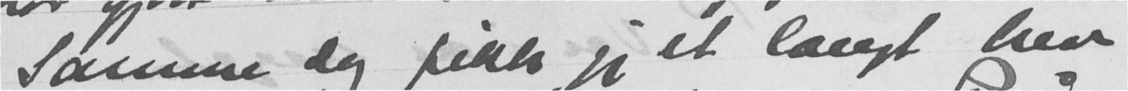Samme dig fikk jeg et langt brev
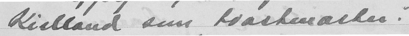Kielland som toastmaster.
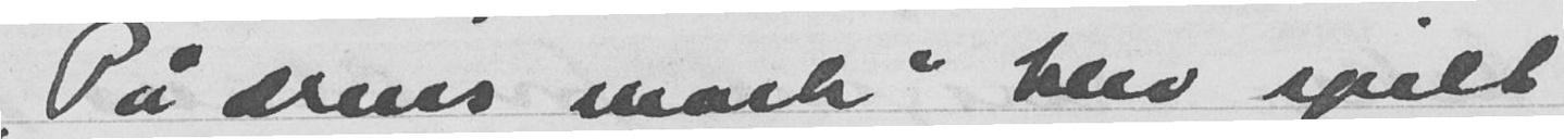"På ærens mark" blev spilt
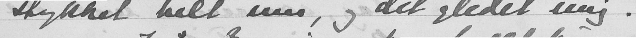stykket helt inn, og det gledet mig.
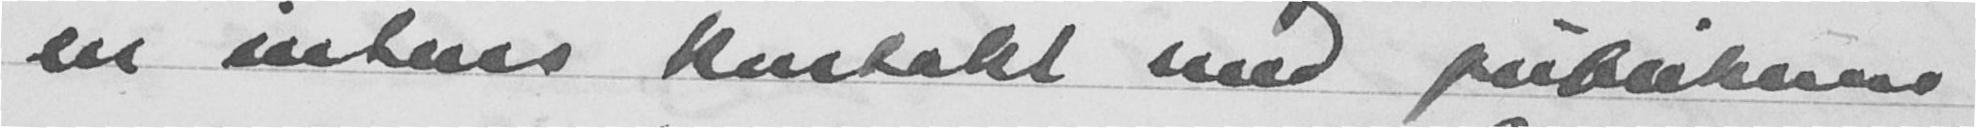en intens kontakt med publikum
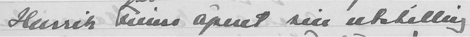Henrik Finne åpnet sin utstilling
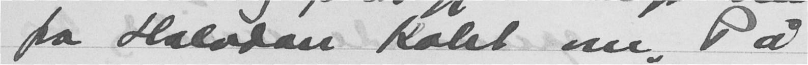fra Halvdan Koht om "På
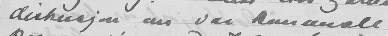diskusjon om var kommende
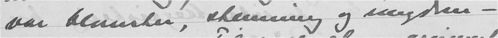var blomster, stemning og ungdom -
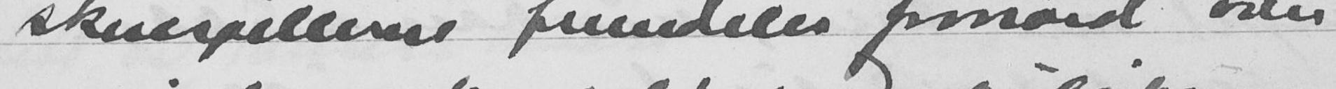skuespillerne fremdeles fornøid over
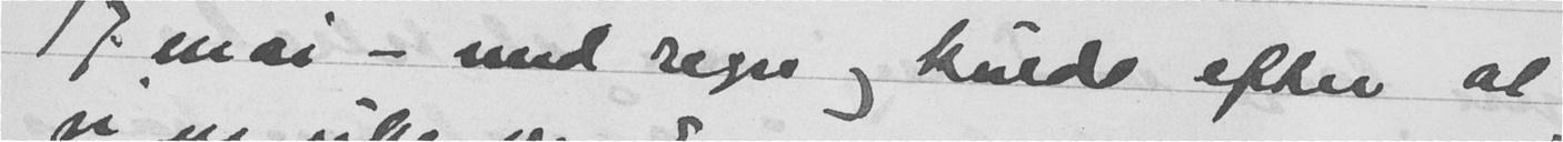17 mai - med regn og kulde efter at
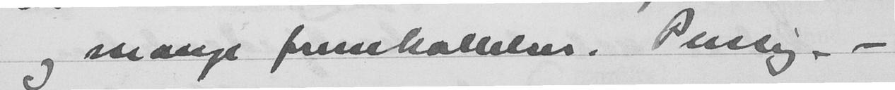og mange fremkallelser. Pussig. -
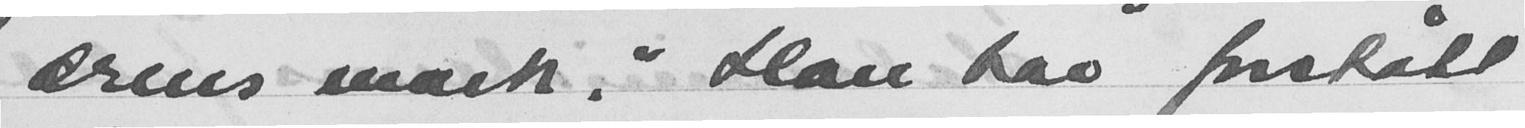ærens mark." Han har forstått
